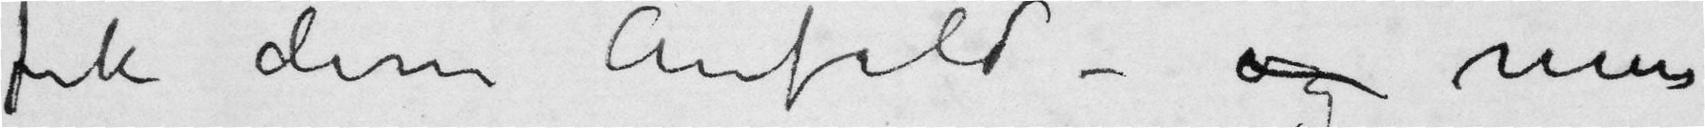fik disse Anfald – og men
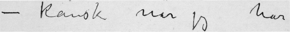– Kanske når jeg har
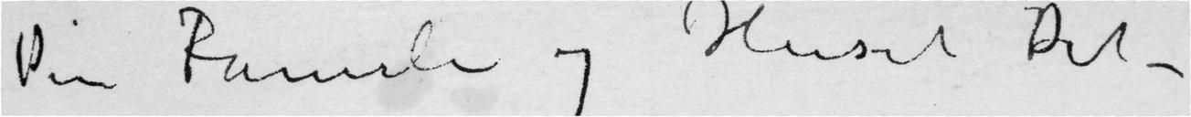Din Familie og Huset Dit –
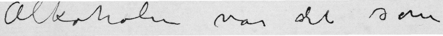Alkoholen var det som
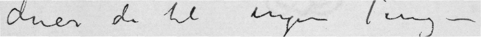duer da til ingen Ting –
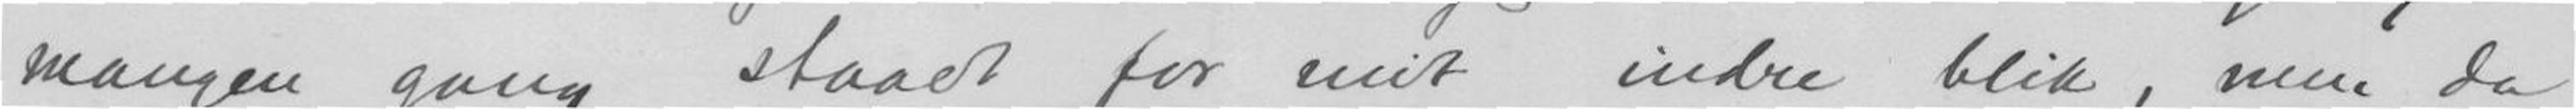mangen gang staaet for mit indre blik, men da
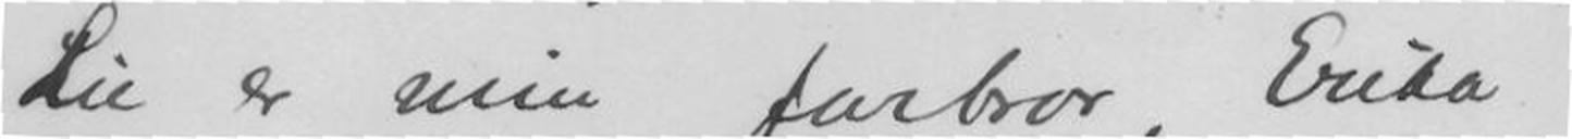Lie er min farbror, Erika
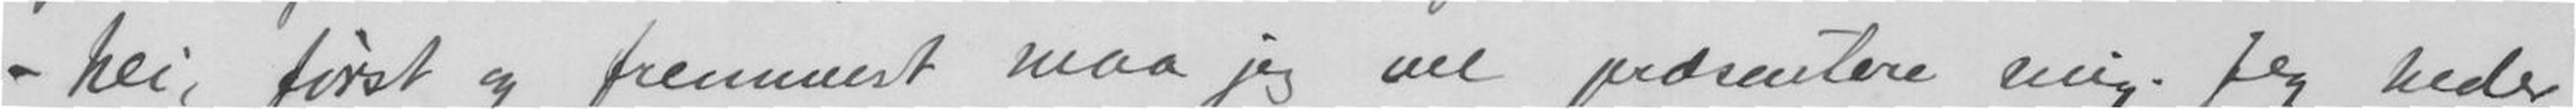- Nei, først og fremmst maa jeg vel presentere mig. Jeg heder
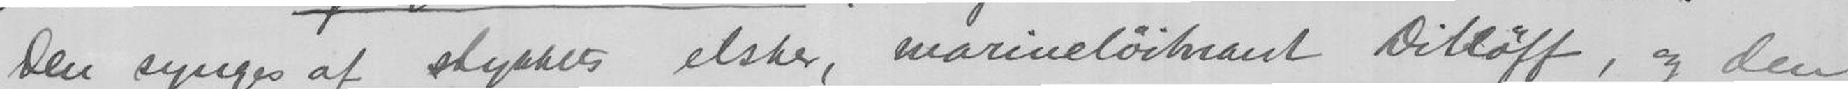Den synges af stykkets elsker, marineløitnant Ditløff, og den
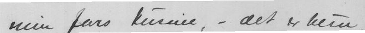min fars kusine, - det er hun
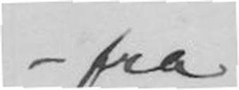- fra
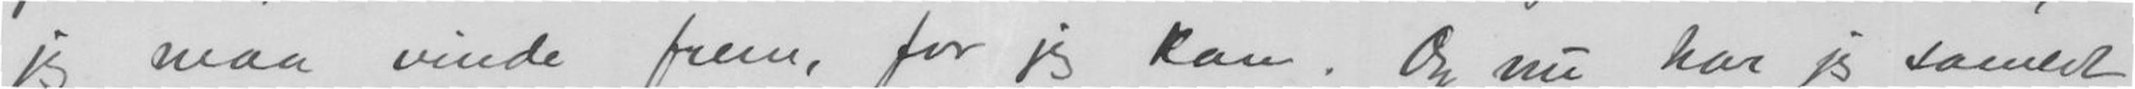jeg maa vinde frem, for jeg kan. og nu har jeg samlet
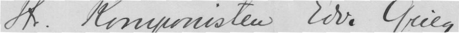Hr. Komponisten Edv. Grieg
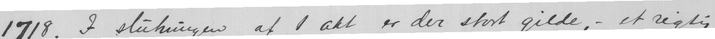1718. I slutningen af 1 akt er der stort gilde, - et rigtig
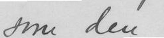som den
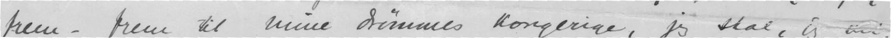frem - frem ti mine drømmers kongerige, jeg skal, jeg vil,
